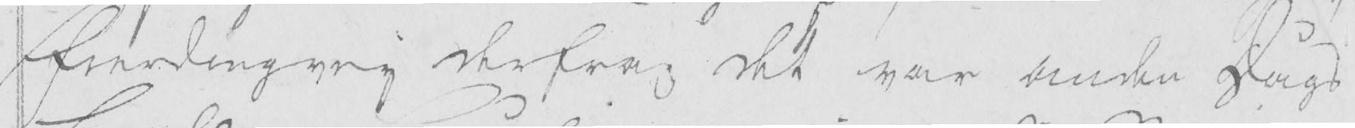Fierdingevej derfra; det var anden Dags
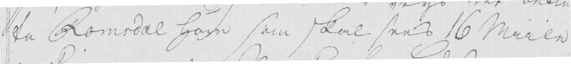te Romsdal Horn som skal sees 16 Miile
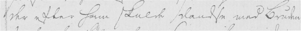der efter ham skulde dandse med Bruden
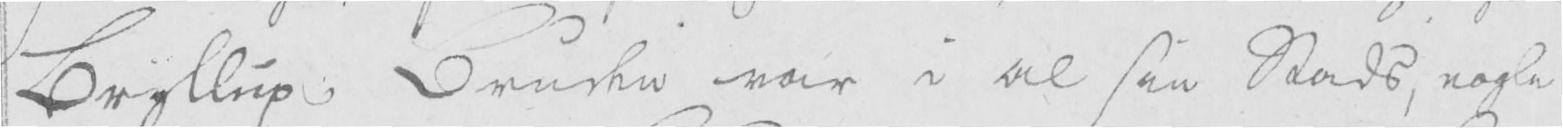Bryllup; Bruden var i al sin Stads, nogle
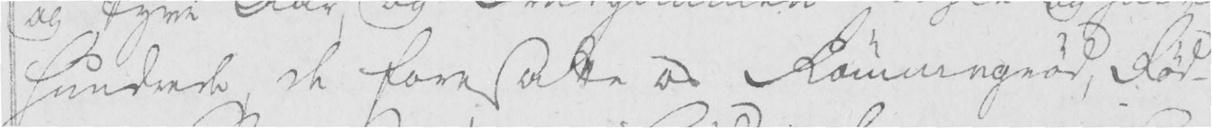hundrede, de foresatte os Kammeregrød, Rød-
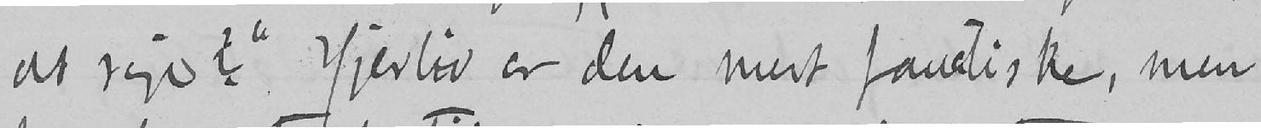at sige?» Hjerløv er den mest fanatiske, men
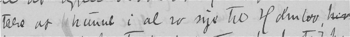telse at kunne i al ro sige til Holmbo, hvr
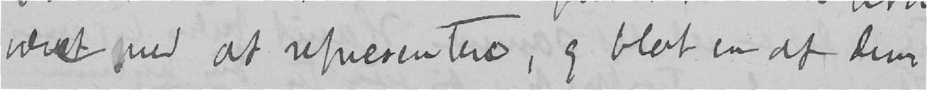været med at representere, og blot en af dem
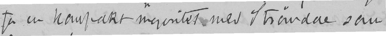for en kompakt majoritet med Strømdal som
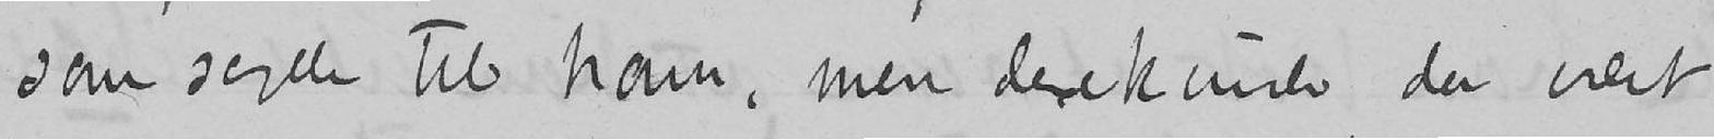som sagde til ham, men der kunde da vært
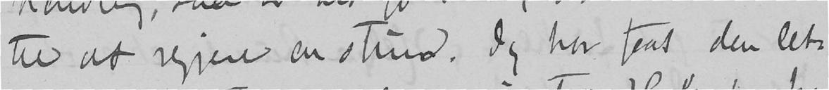til at regjere en stund. Jeg har faat den let-
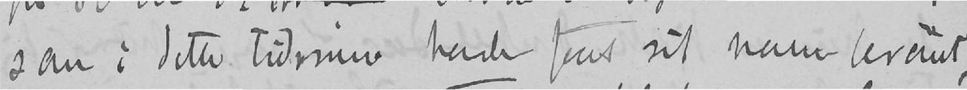som i dette tidsrum havde faat sit navn berømt,
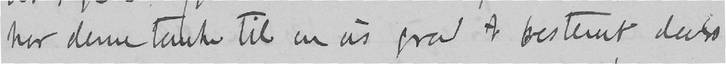har denne tanke til en vis grad t bestemt deres
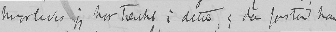hvorledes jeg har tænkt i dette, og da forstod han
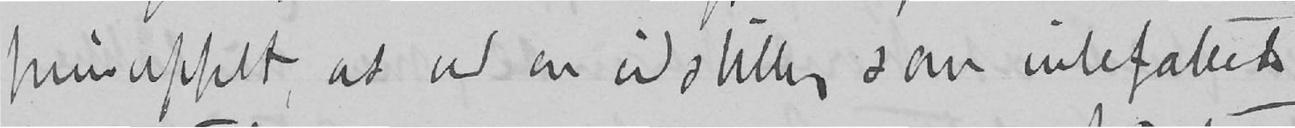prinsippet, at ved en udstilling som inbefattede
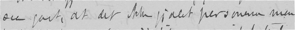saa godt, at det ikke gjaldt personene men
